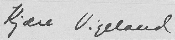Kjære Vigeland
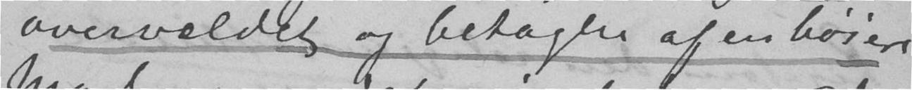overveldet og betagen af en høiere
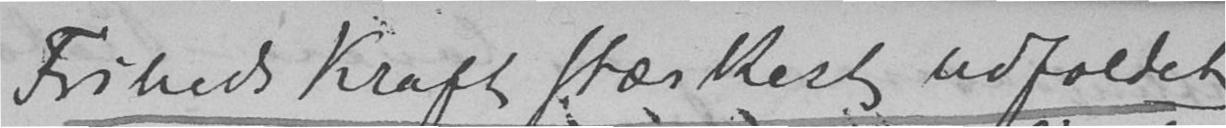Friheds Kraft stærkest udfoldet,
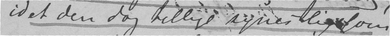idet den dog tillige synes ligesom
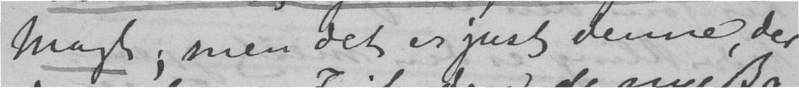Magt, men det er just denne, der
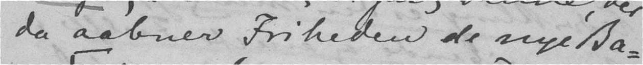da aabner Friheden de nye Ba-
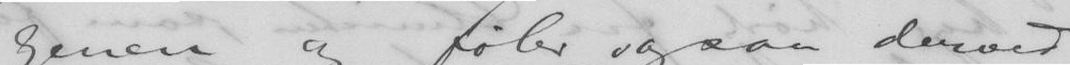genen og føler ogsaa derved
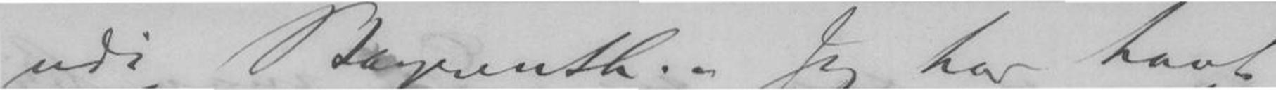udi Bayreuth. - Jeg har havt
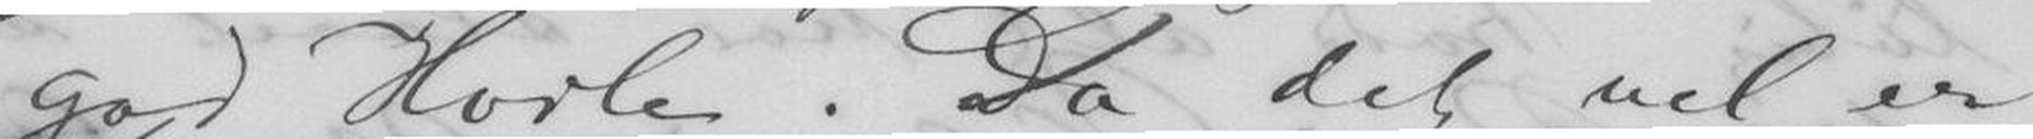god Hvile. Da det vel er
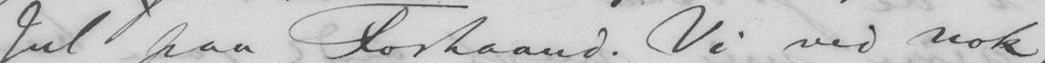Jul" paa Forhaand. Vi ved nok,
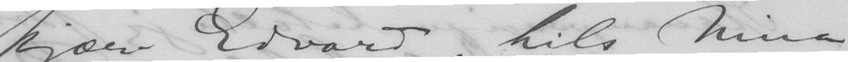kjære Edvard, hils Nina
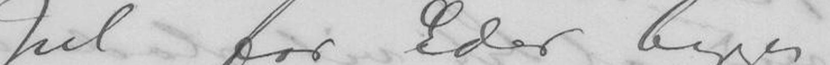Jul for Eder begge!
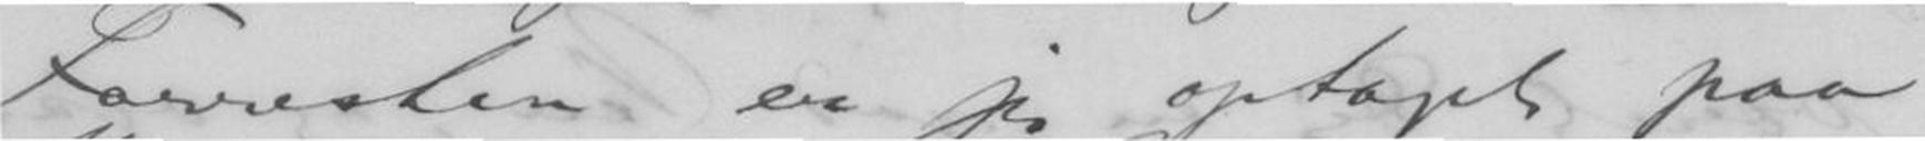Forresten er jeg optaget paa
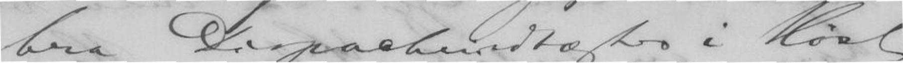bra Dispacheindtægter i Høst.
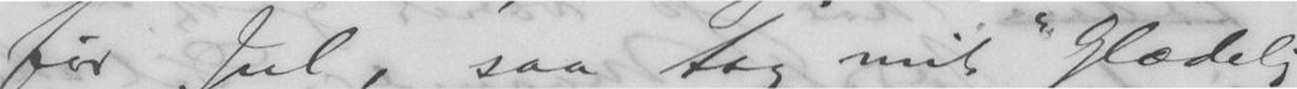før Jul, saa tag mit "Glædelig
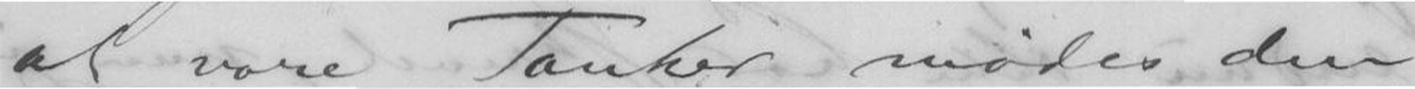at vore Tanker mødes den
